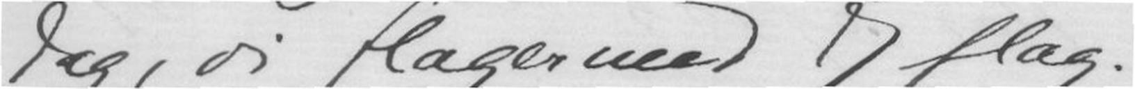dag, vi flager med 7 flag.
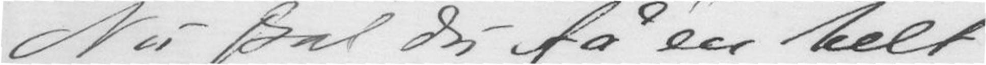Nu skal du få en helt
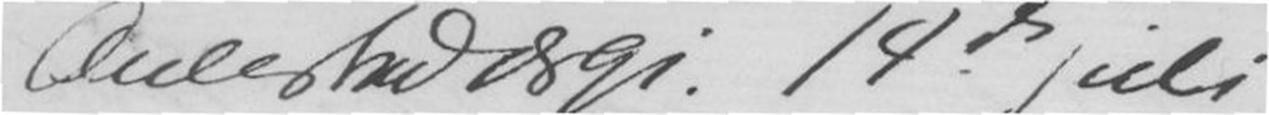Aulestad 1891. 14de juli
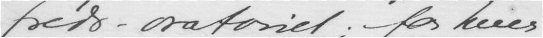freds-oratoriet; for hver
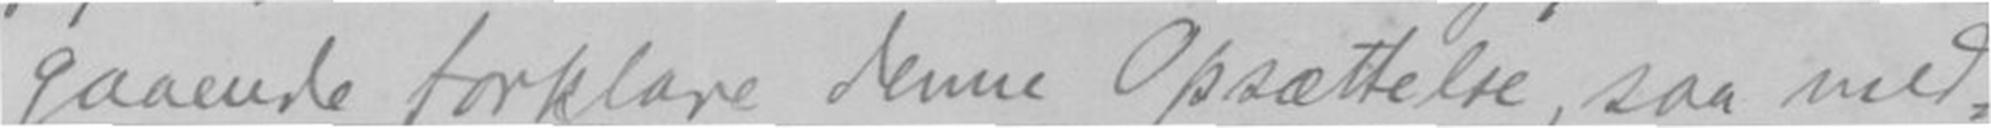gaaende forklare denne Opsættelse, saa med-
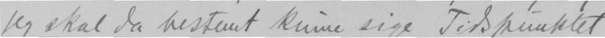jeg skal da bestemt kunne sige Tidspunket
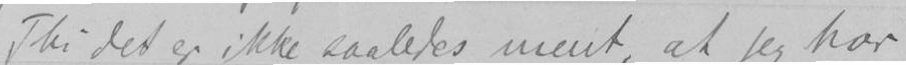Thi det er ikke saaledes ment, at jeg har
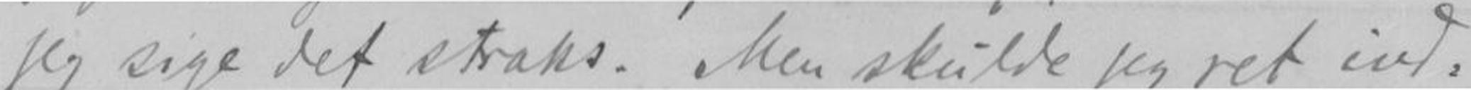jeg sige det straks. Men skulde jeg ret ind-
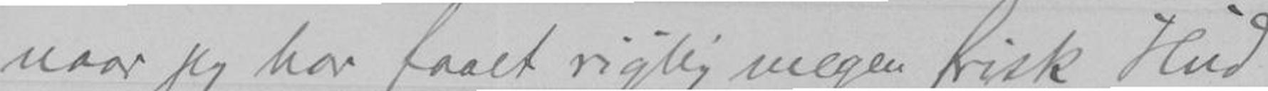naar jeg har faaet rigtig megen frisk Hud
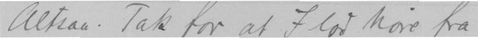Altsaa Tak for I lod høre fra
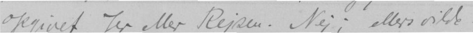opgivet Jer eller Rejsen. Nej; ellers vilde
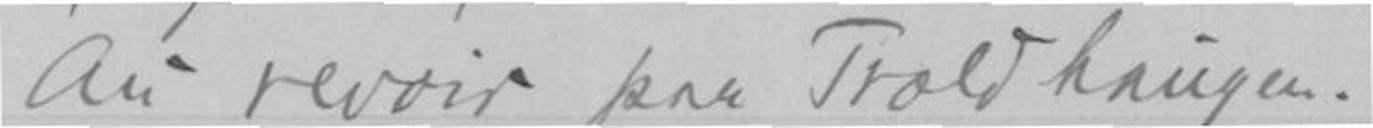Au revoir paa Troldhaugen.
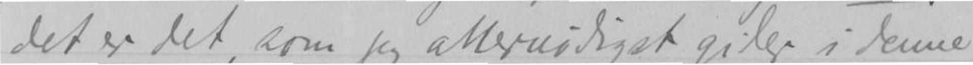det er det, som jeg allernødigst gider i denne
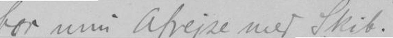for min Afrejse med Skib.
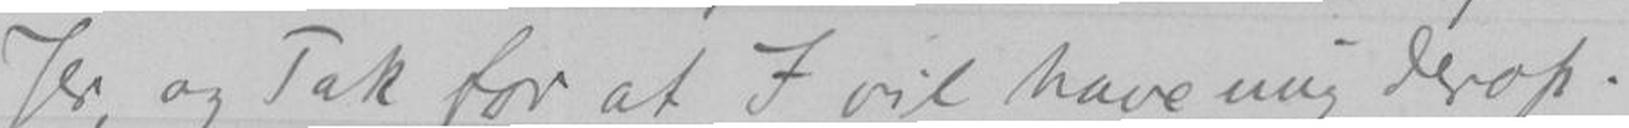Jer, og Tak at I vil have mig derop.
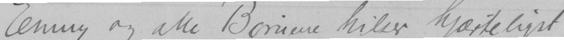Emmy og alle Børnene hilser hjærteligst
